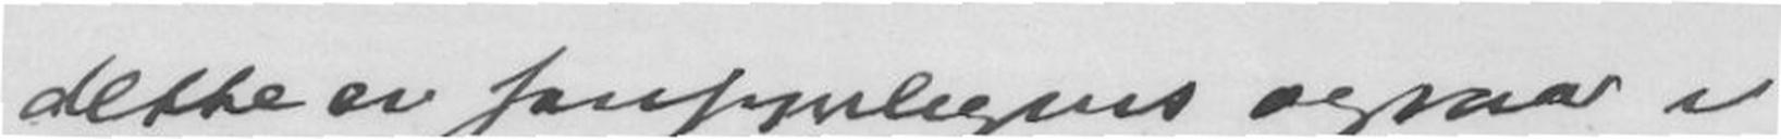Dette er sansynligvis ogsaa i
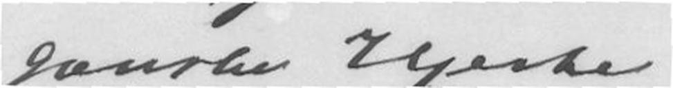ganske Hjerte
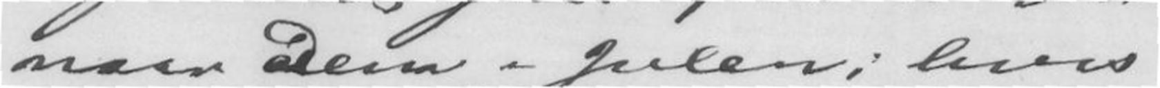naar Dem i Julen; hvis
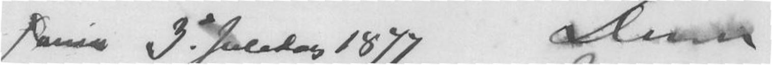Xania 3d Juledag 1877 Deres
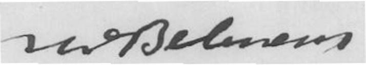Joh D Behrens
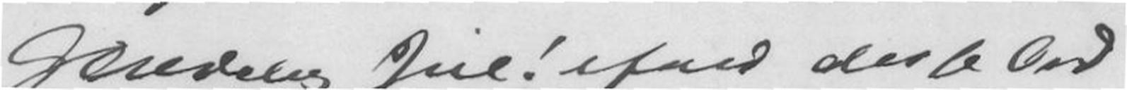Glædelig Jul! ifald disse Ord
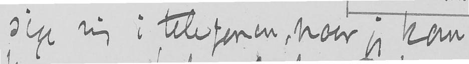sige mig i telefonen, naar jeg kan
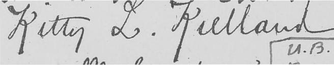Kitty L. Kielland
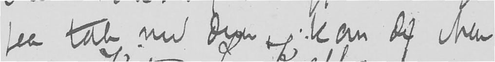faa tale med dem. kom de ikke
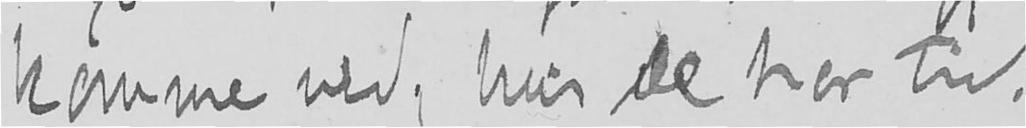komme ned, hvis de har tid.
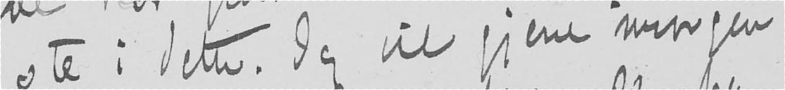ste i dette. Jeg vil gjerne imorgen
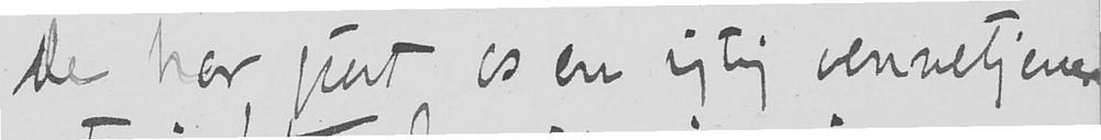de har giort os en rigtig vennetjene-
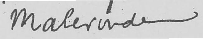Malerinde
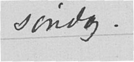søndag.
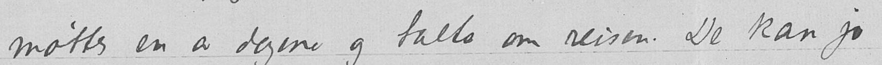møttes en av dagene og talte om reisen. De kan jo
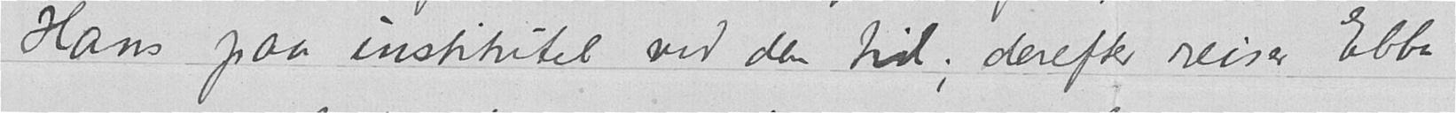Hans paa institutet ved den tid; derefter reiser Ebba
